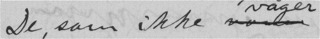De, som ikke
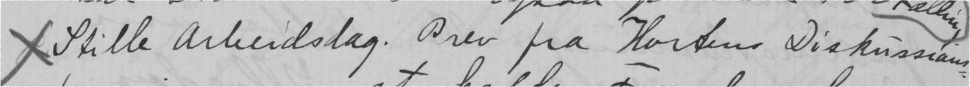x Stille Arbeidsdag. Brev fra Hortens Diskussions-
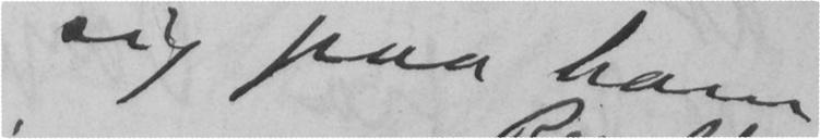sig paa ham
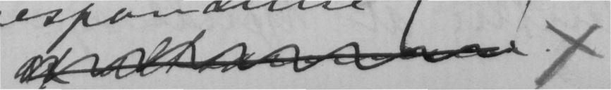. x
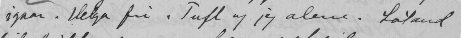igaar. Helga fri . Tuft og jeg alene. Løland
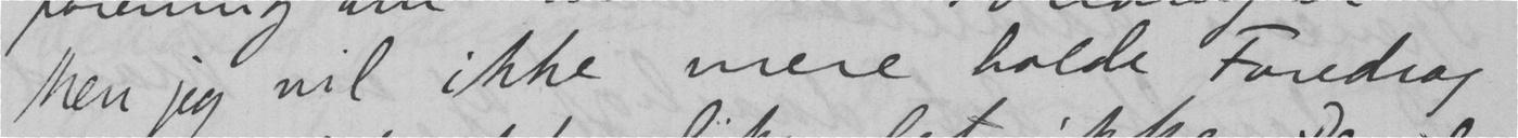Men jeg vil ikke mere holde Foredrag
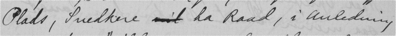Plads, Snedkere  ha Raad, i Anledning
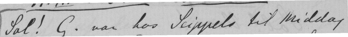Sol! G. var hos Seippels til middag
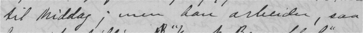til middag; men han arbeider, saa
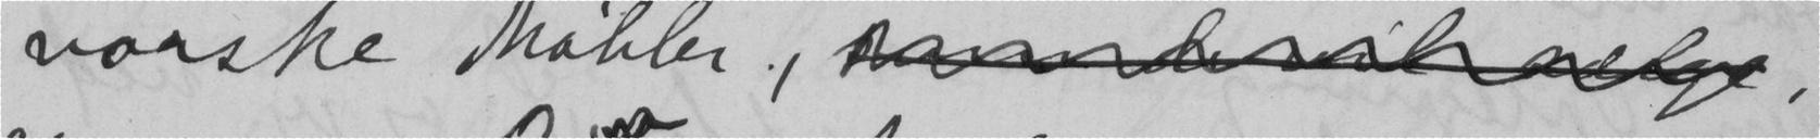norske møbler., ,
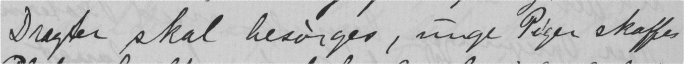Dragter skal besørges, unge Piger skaffes
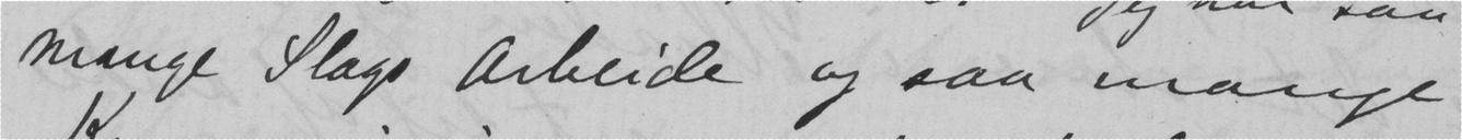mange Slags arbeide og saa mange
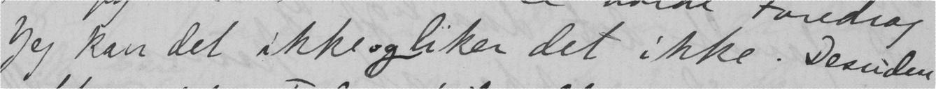Jeg kan det ikkes og liker det ikke. Desuden
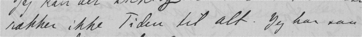rækker ikke Tiden til alt. Jeg har saa
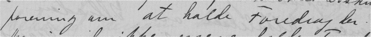forening om at holde Foredrag der.
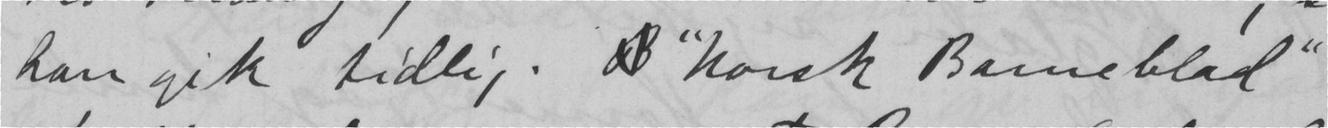han gik tidlig.  "norsk Barneblad"
